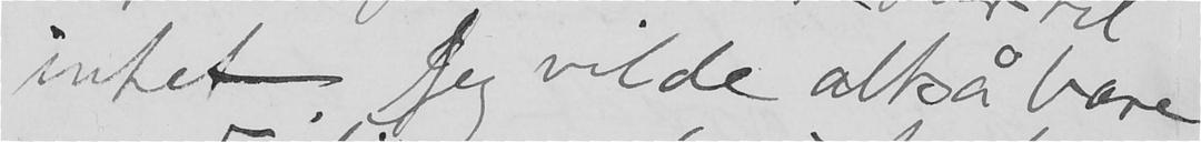intet. Jeg vilde altså bare
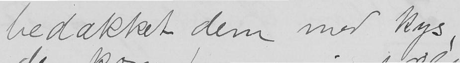bedækket dem med kys,
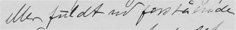eller fuldt ud forstående
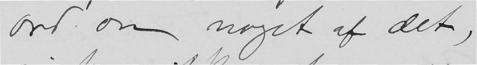ord om noget af det,
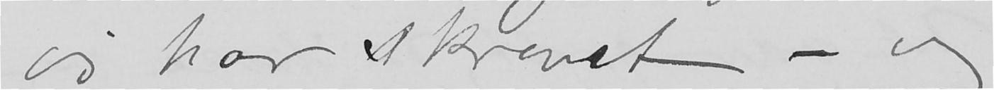jeg har skrevet - og
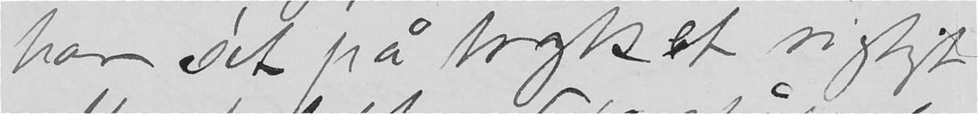har sét på tryk et rigtigt
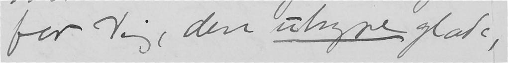for Dig, den uhyre glæde,
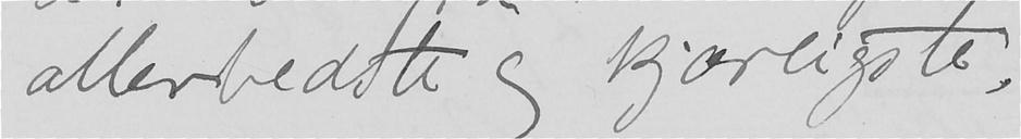allerbedste og kjærligste.
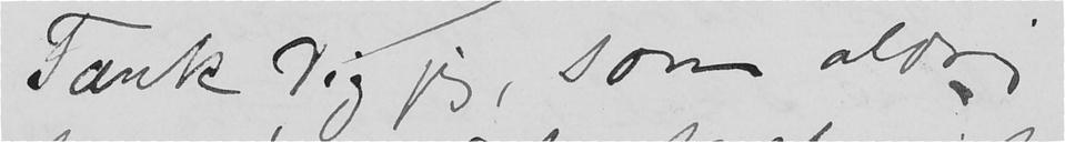Tænk Dig jeg, som aldrig
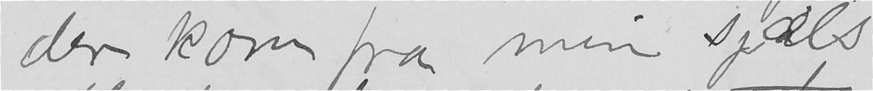der kom fra min sjæls
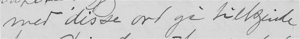med disse ord gi tilkjende,
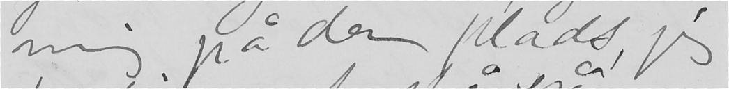mig på den plads, jeg
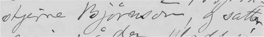stjerne Bjørnson, og sætter
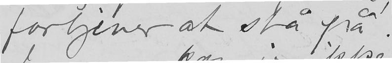fortjener at stå på.
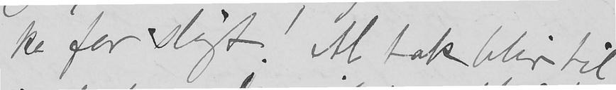ke for sligt! Al tak blir til
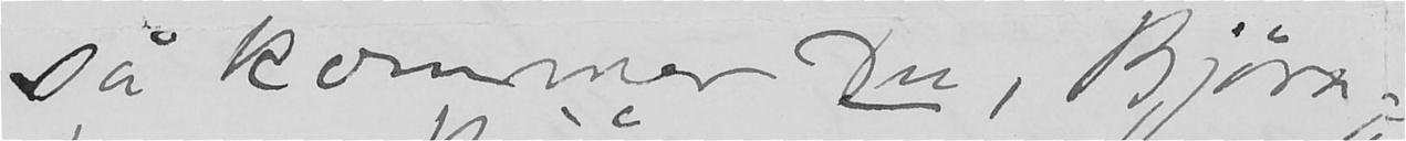så kommer Du, Bjørn-
